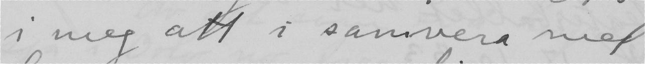i meg att i samvera med
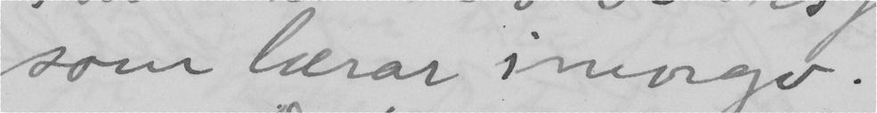som lærar imorgo.
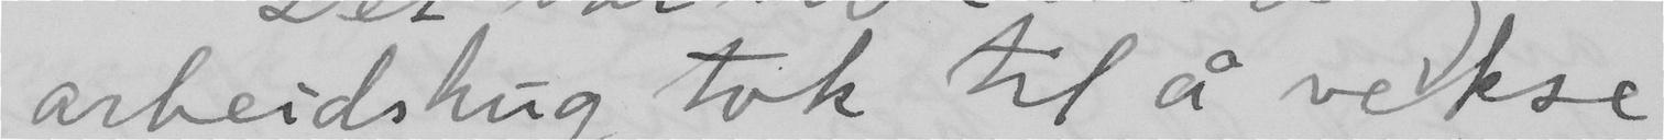arbeidshug tok til å vekse
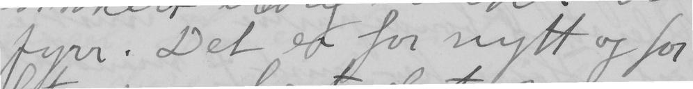fyrr. Det er for nytt og for
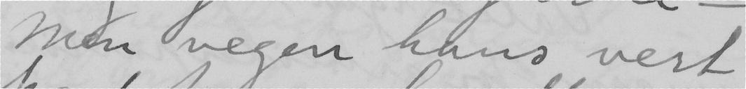Men vegen hans vert
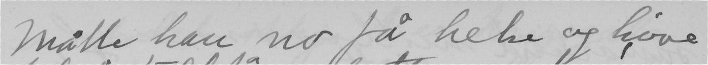Måtte han no få helse og höve
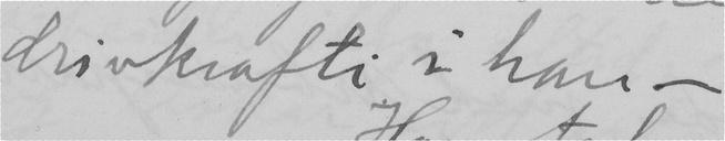drivskrafti i han –
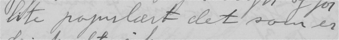lite populært det som er
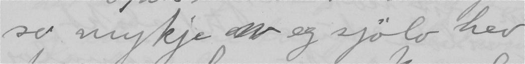so mykje  eg sjölv hev
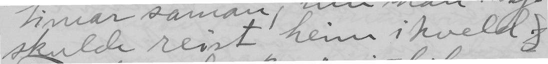skulde reist heim ikveld.
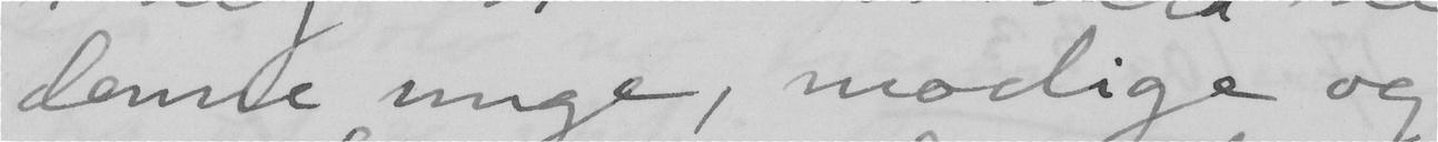desse unge, modige og
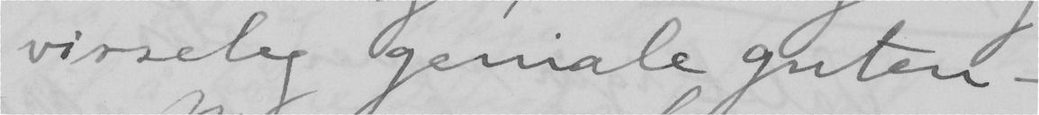visseleg geniale guten –
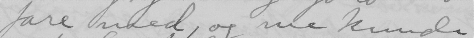fare med, og me kunde
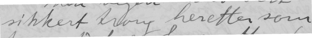sikkert trong heretter som
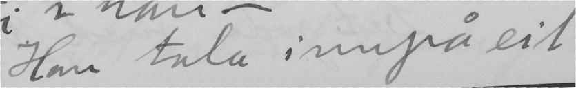Han tala innpå eit
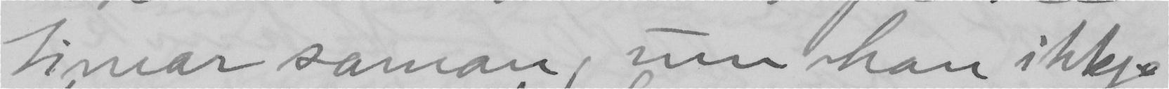timar saman, um han ikkje
